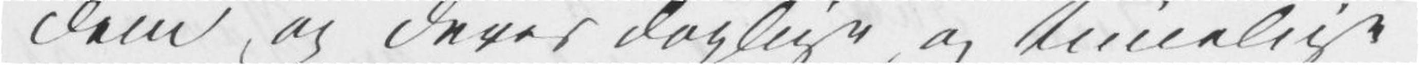dem og deres daglige og timelige
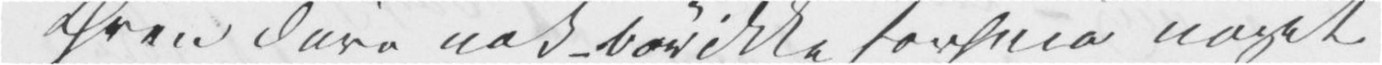Øren døve nok – bør ikke forsmå noget
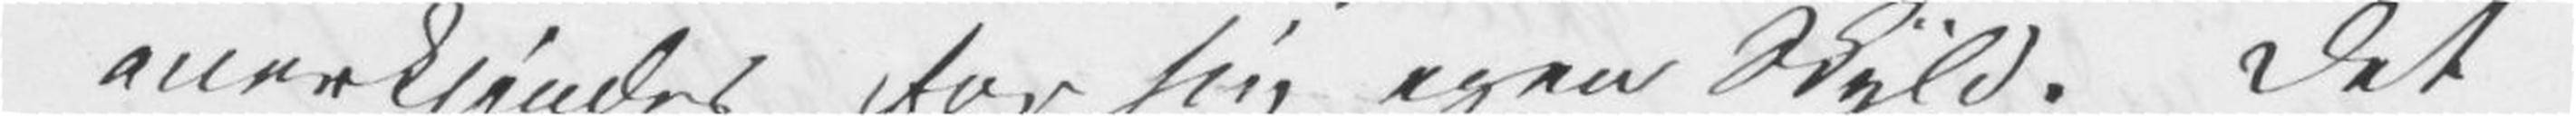anerkjendes for sin egen Skyld. Det
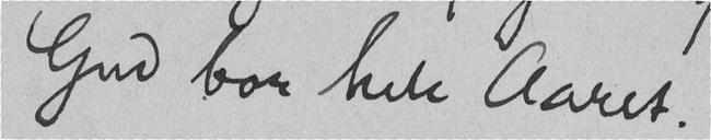Gud bor hele Aaret.
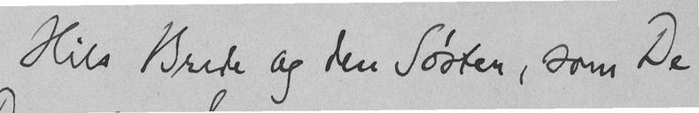Hils Brede og den Søster, som De
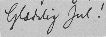Glædelig Jul!
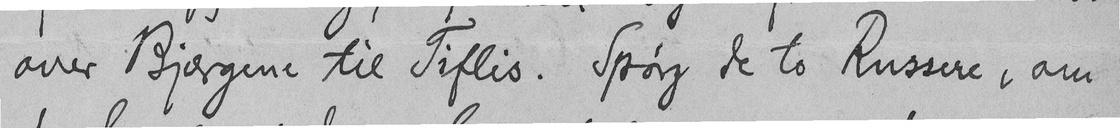over Bjærgene til Tiflis. Spørg de to Russere, om
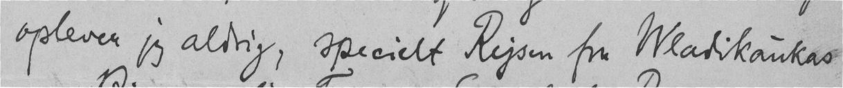oplever jeg aldrig, specielt Rejsen fra Wladikaukas
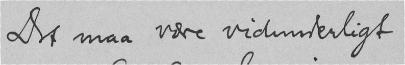Det maa være vidunderligt
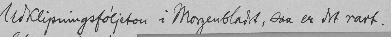Udklipningsføljeton i Morgenbladet, saa er det rart.
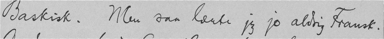Baskisk. Men saa lærte jeg jo aldrig Fransk.
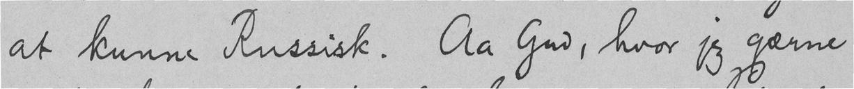at kunne Russisk. Aa Gud, hvor jeg gærne
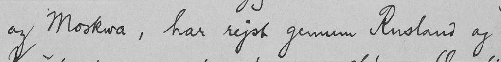og Moskva, har rejst gennem Rusland og
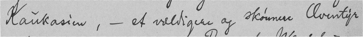Kaukasien, - et vældigere og skønnere Æventyr
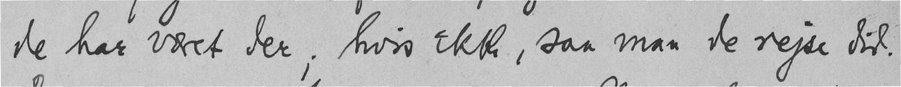de har været der; hvis ikke, saa maa de rejse did.
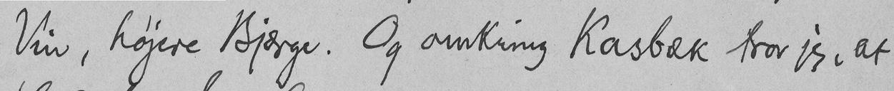Vin, højere Bjærge. Og omkring Kasbæk tror jeg, at
